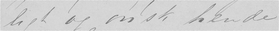ligt og ønsk hende
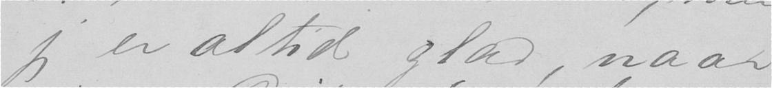jeg er altid glad, naar
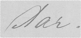Aar.
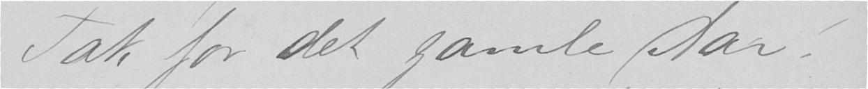Tak for det gamle Aar!
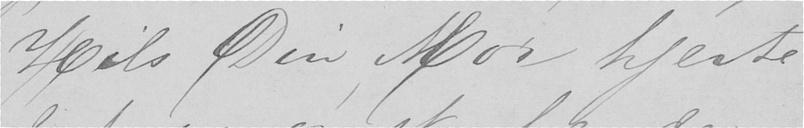Hils Din Mor hjerte-
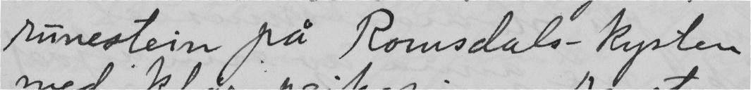runestein på Romsdals-kysten
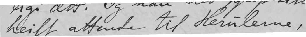heilt attende til Herulerne,
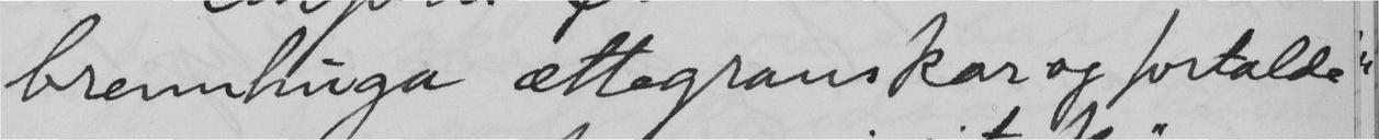brennhuga ættegranskar og fortalde
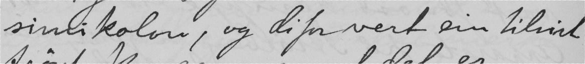simikolon, og difor vert ein tilsist
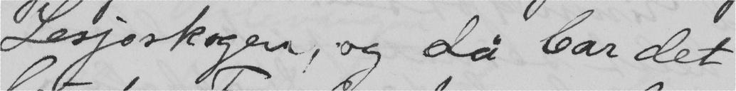Lesjoskogen, og då bar det
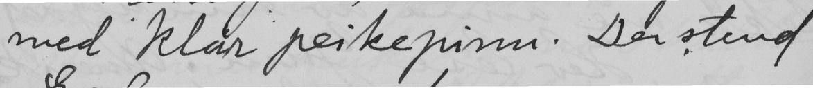med klår peikepinn. Der stend
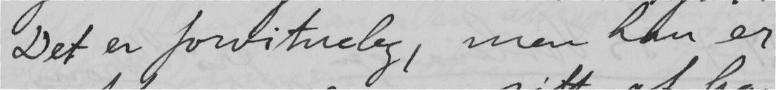Det er forvitneleg, men han er
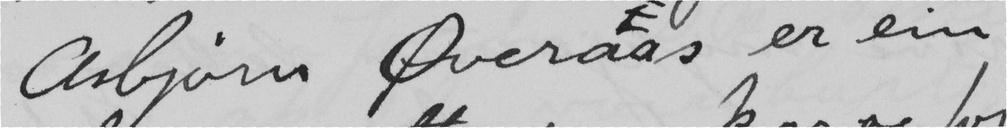Asbjörn Øveraas er ein
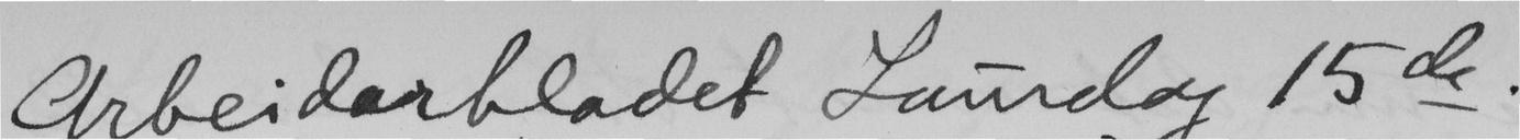Arbeidarbladet Laurdag 15de.
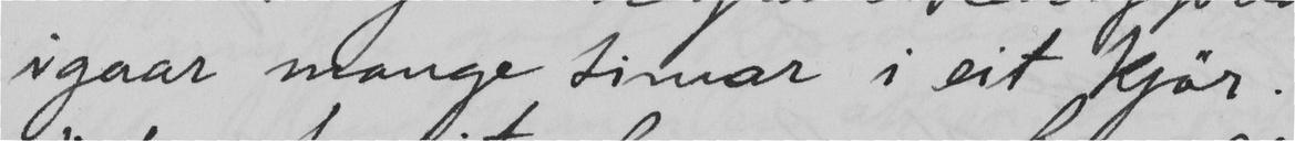igaar mange timar i eit kjör.
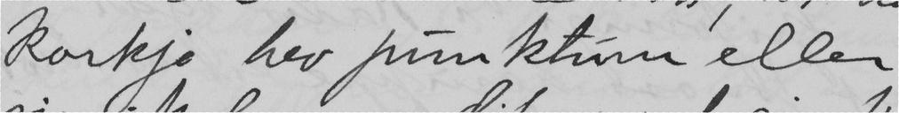korkje hev punktum eller
